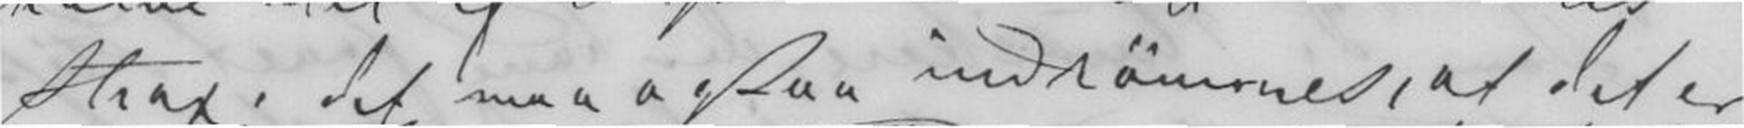strax; det maa ogsaa indrømmes, at det er
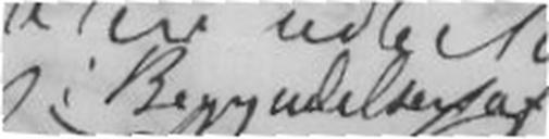Begyndelsen af
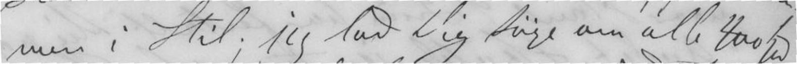men i Stil; jeg lod Dig søge om alle 400 Spd
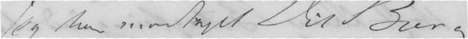Jeg har modtaget Dit Brev og
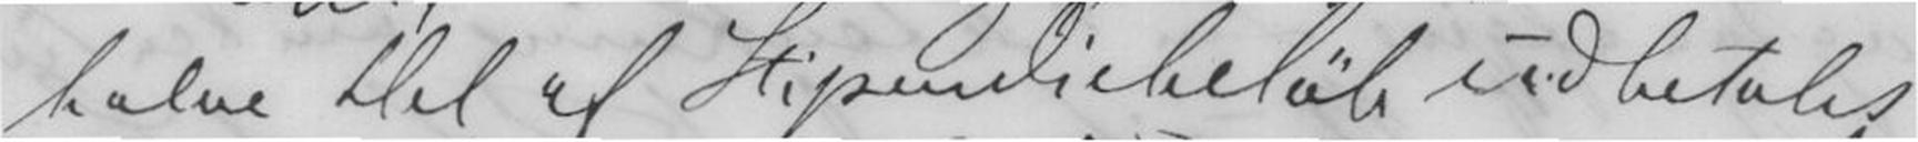halve Del af Stipendiebeløb udbetales
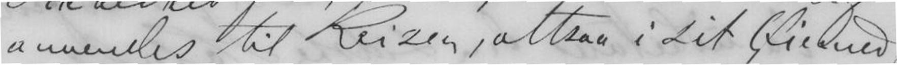anvendes til Reisen, altsaa i sit Øiemed
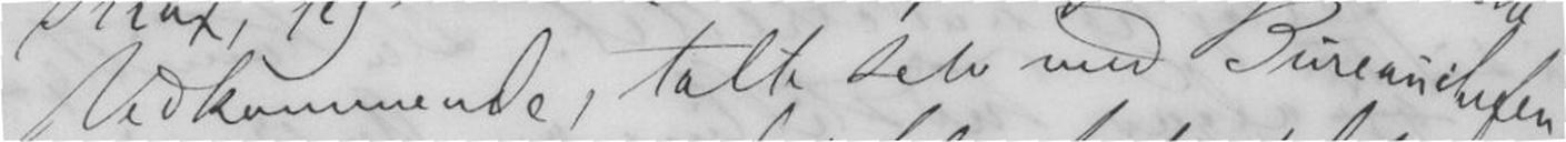Vedkommende; talte selv med Bureauchefen
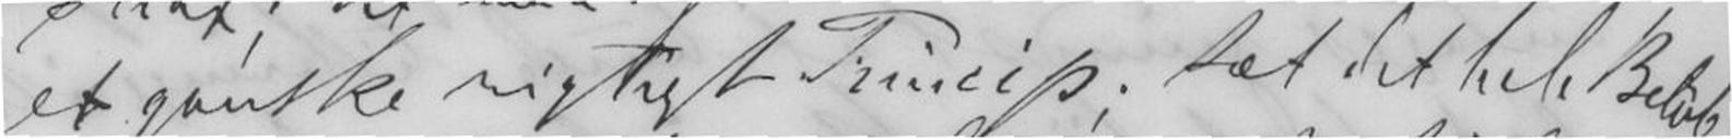et ganske vigtig Prinsip; sæt det hele Beløb
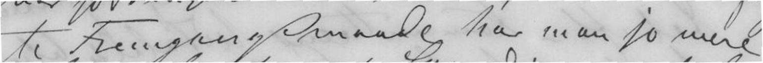te Fremgangsmaade har man jo mere
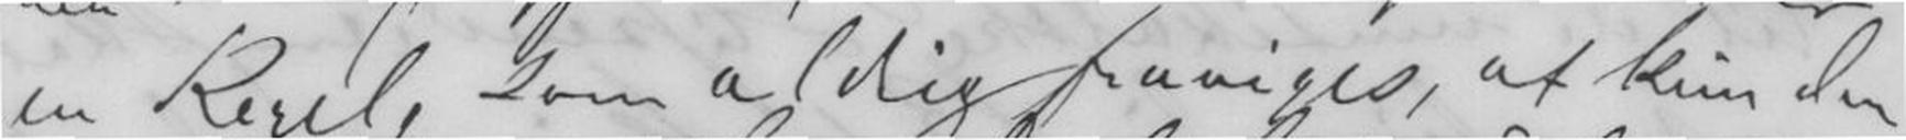en Regel, som aldrig fraviges, at kun den
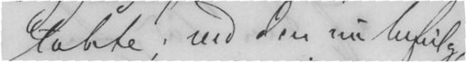tabte; ved den nu befulg-
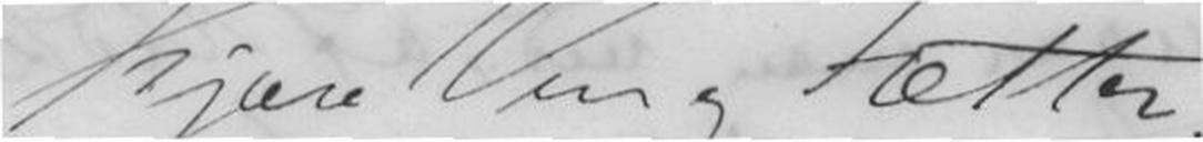Kjære Ven og Fætter.
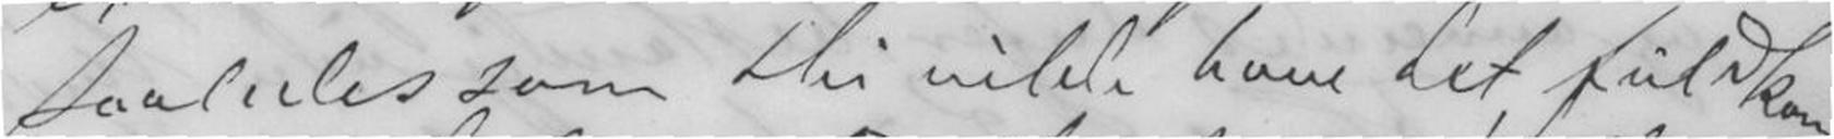saaledes som Du vilde have det, fuldkom-
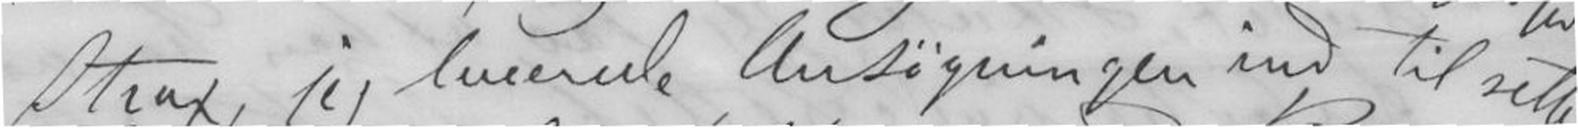strax, jeg leverede Ansøgningen ind til rette
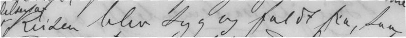Reisen blev syg og faldt fra, saa
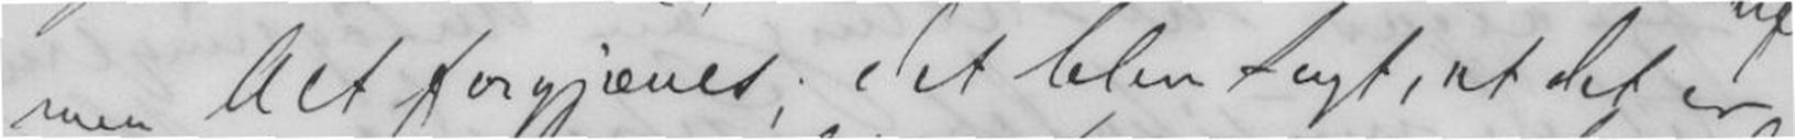men Alt forgjæves; det blev sagt, at det er
